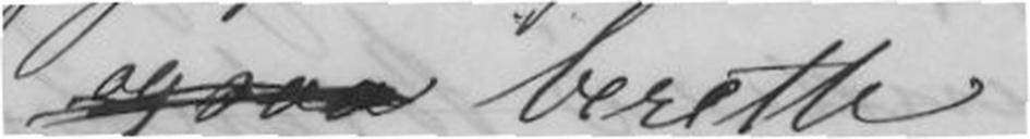ogsaa berette.
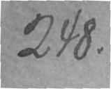248.
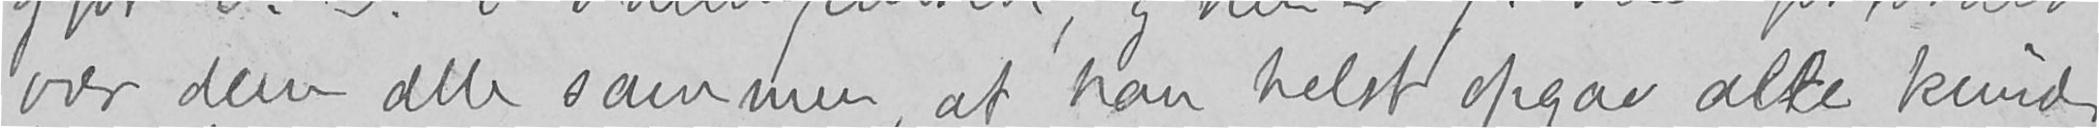over dem alle sammen, at han helst opgav alle kvinder,
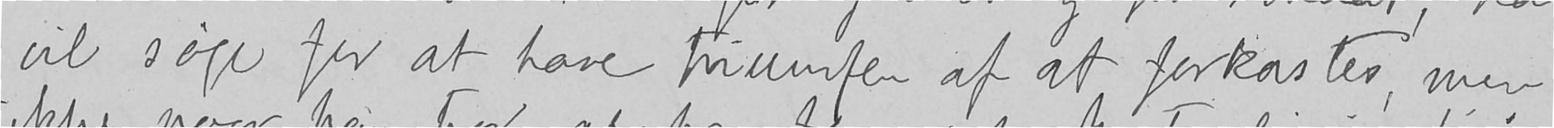vil søge for at have triumfen af at forkastes, men
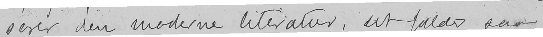serer den moderne literatur, det falder saa
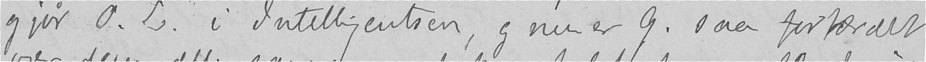gjør O. L. i Intelligentsen, og nu er G. saa forfærdet
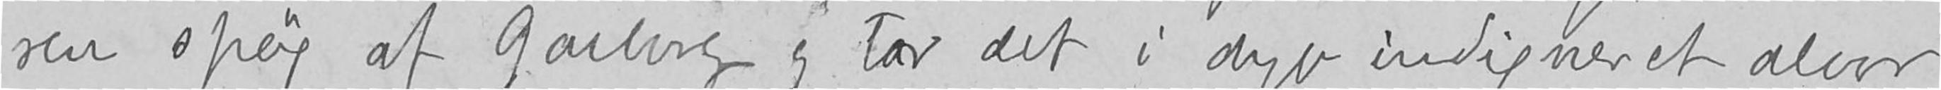ren spøg af Garborg og tar det i dyb indigneret alvor,
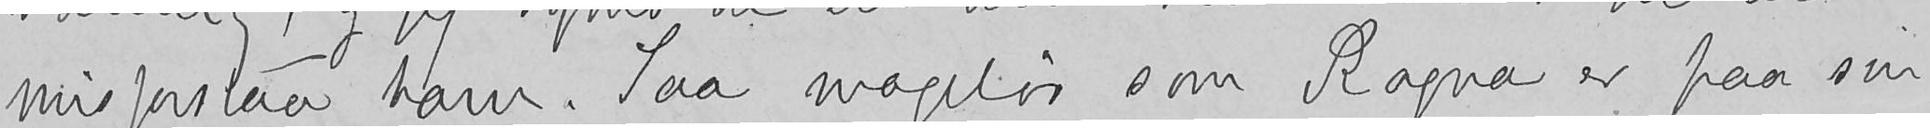misforstaa ham. Saa mageløs som Ragna er paa sin
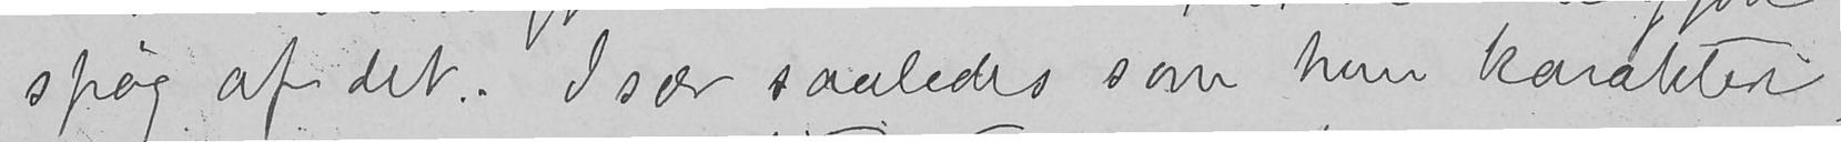spøg af det. Især saaledes som hun karakteri-
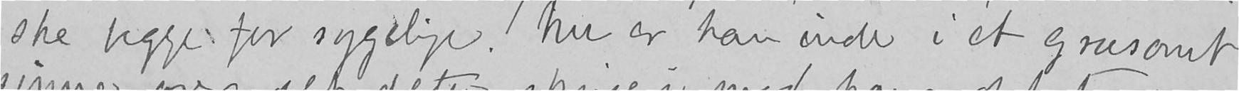ske begge for sygelige. Nu er han inde i et grusomt
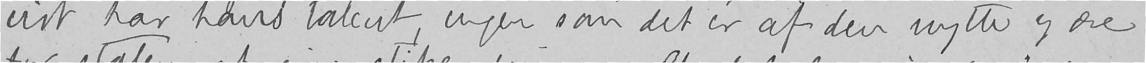vist har hans talent, ingen som det er af den nytte og ære
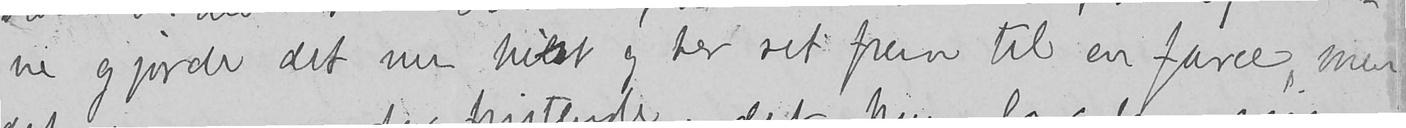ne gjorde det nu hist og her ret frem til en farce, men
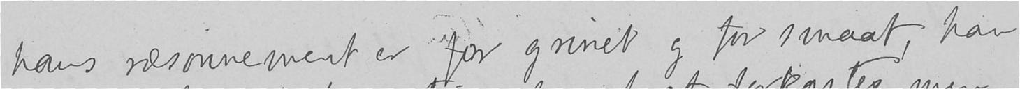hans ræsonnement er for grinet og for smaat, han
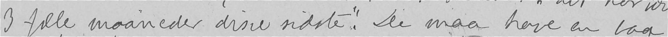3 fæle maaneder disse sidste." De maa have en bag-
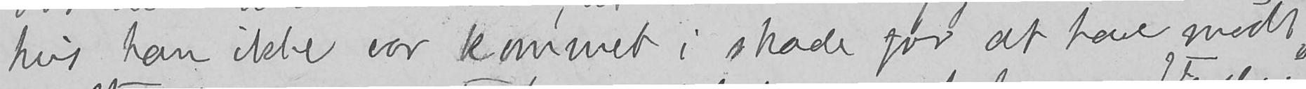hvis han ikke var kommet i skade for at have mødt
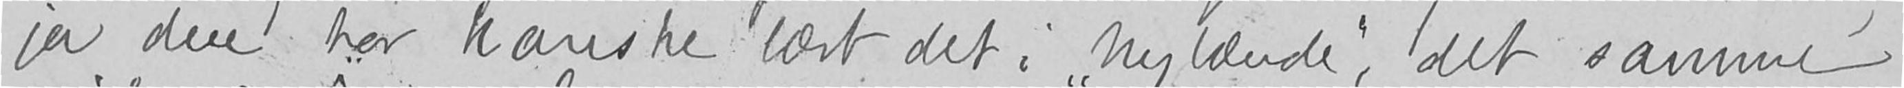ja dere har kanske læst det i Nylænde, det samme
